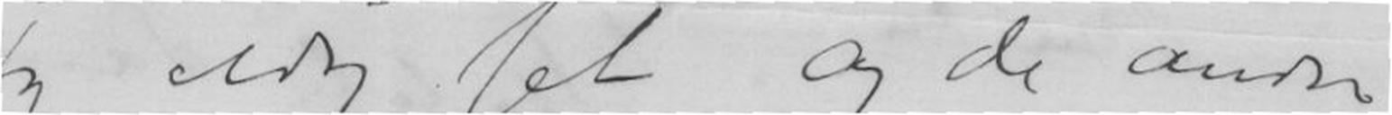jeg aldrig set og de andre
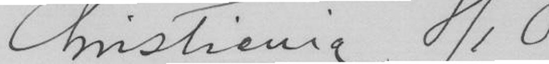Christiania 8/1 07.
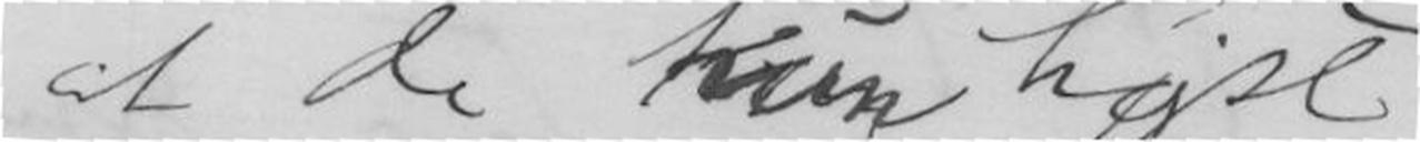at de kun højst
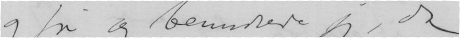og fri og beundrede jeg, de
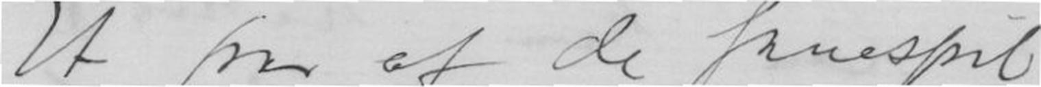Et par af de skuespil-
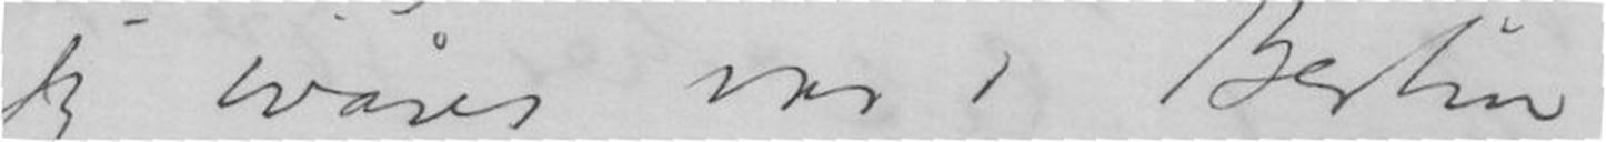jeg wåves nu i Berlin.
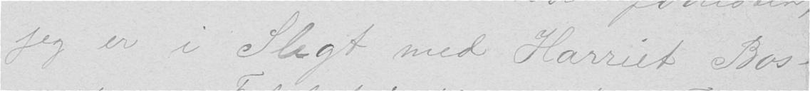jeg er i Slegt med Harriet Bos-
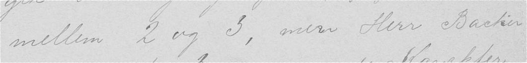mellem 2 og 3, men Herr Backer
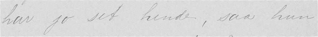har jo set hende, saa hun
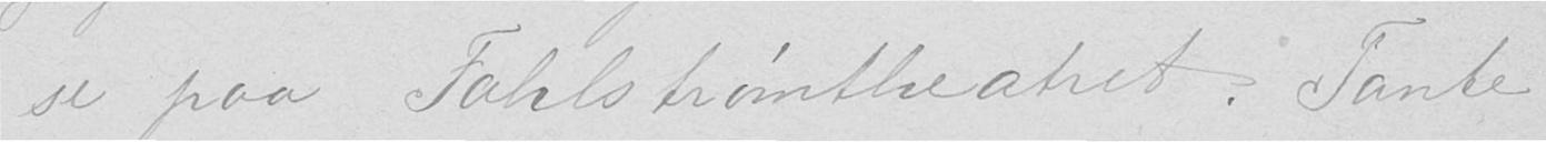se paa Fahlstrømtheatret. Tante
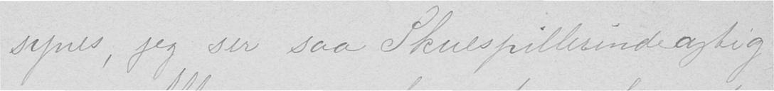synes, jeg ser saa Skuespillerindeagtig
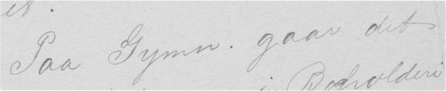Paa Gymn. gaar det
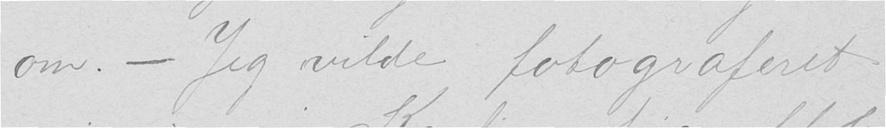om. – Jeg vilde fotograferet
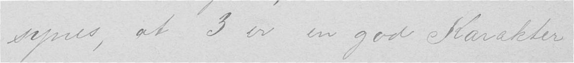synes, at 3 er en god Karakter
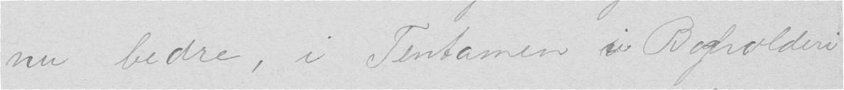nu bedre, i Tentamen i Bogholderi
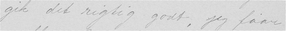gik det rigtig godt, jeg faar
